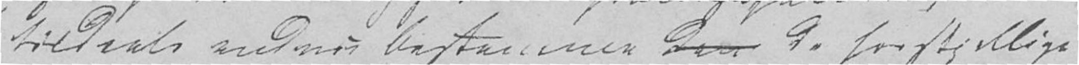tildeels endnu bestemmer den de forskjellige
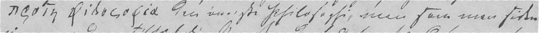XX den øverste Philosophi, men som man siden
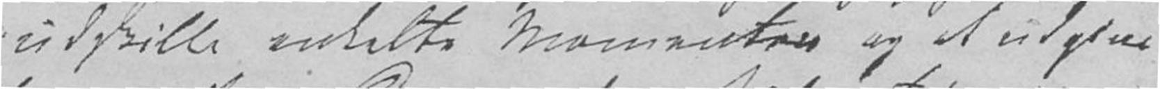udskille enkelte Momenter og at udgive
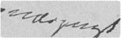nærmest
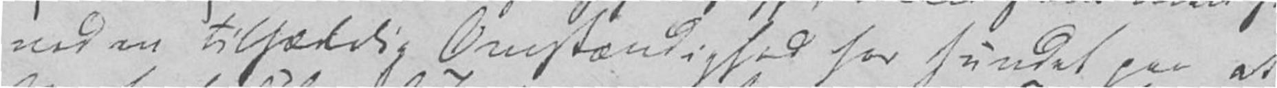ved en tilfældig Omstændighed har fundet paa at
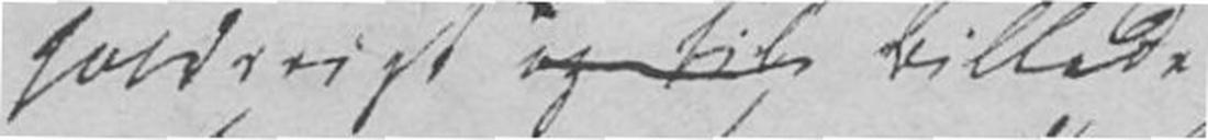holdsrigt Billede
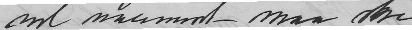vel nærmest maa ske
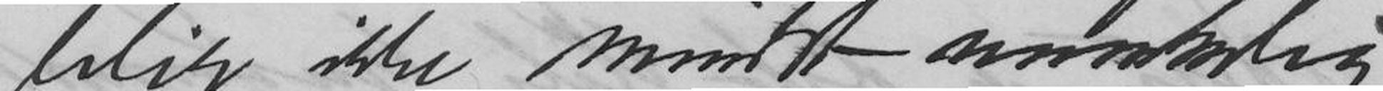blir ikke mindst vanskelig.
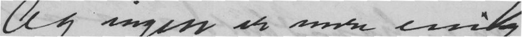Og ingen er mere enig
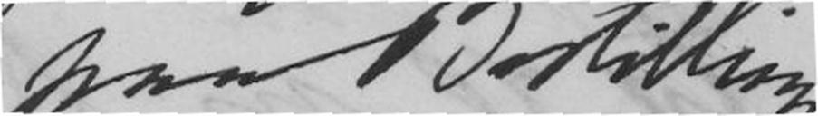paa Bestilling.
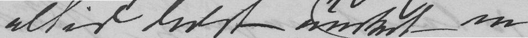altid helst ønsket en
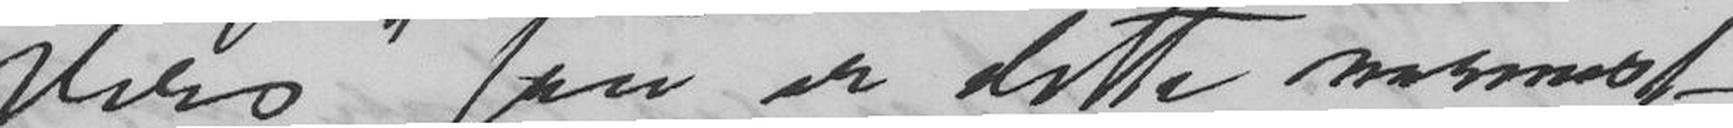Vers" saa er dette nærmest
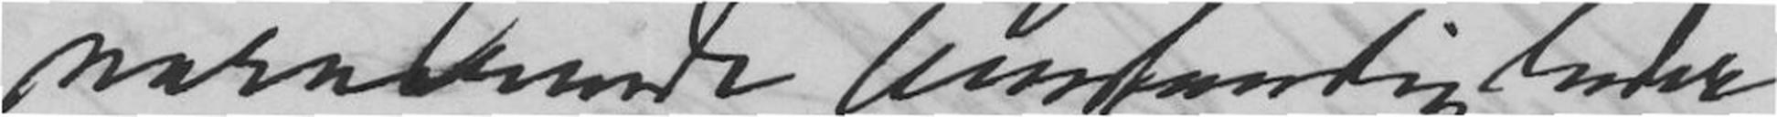nærværende Omstendigheder
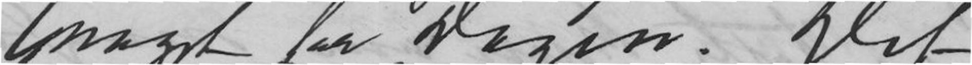noget for Dagen. Det
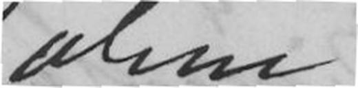alene
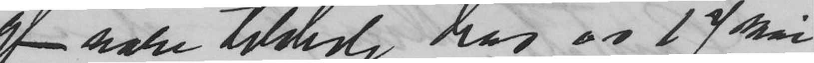at være tilstede hos os 17 Mai
